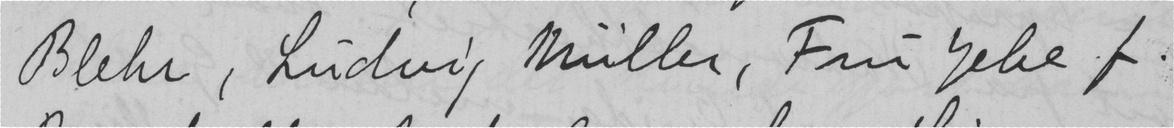Blehr, Ludvig Müller, Fru Jebe f.
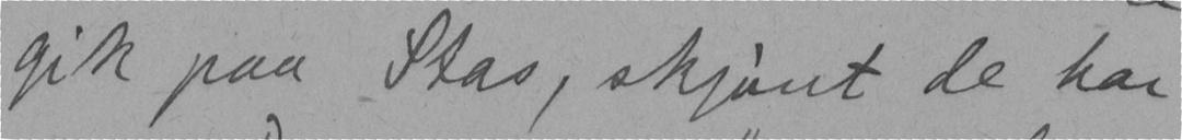gik paa Stas, skjønt de har
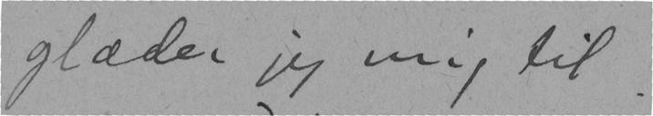glæder jeg mig til.
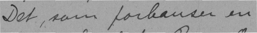Det som forbauser en
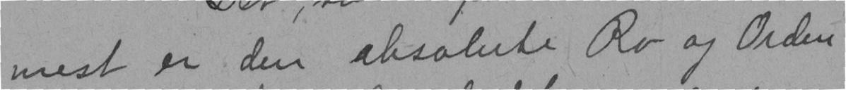mest er den absolute Ro og Orden
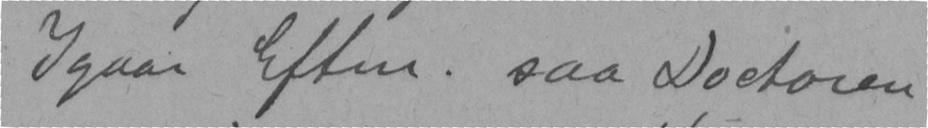Igaar Eftm. saa Doctoren
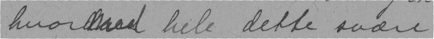hvor hele dette svære
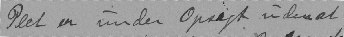Plet er under Opsigt uden at
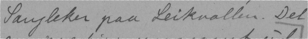Sangleker paa Leikvollen. Det
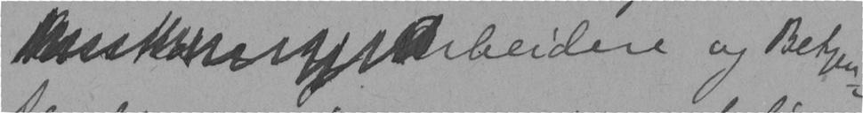arbeidere og Betjen-
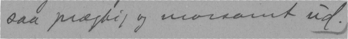saa prægtig og morsomt ud.
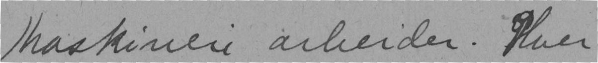Maskineri arbeider. Hver
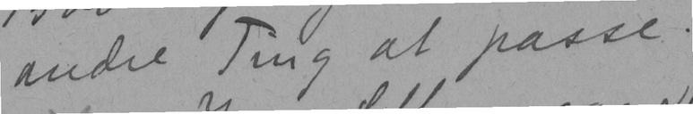andre Ting at passe.
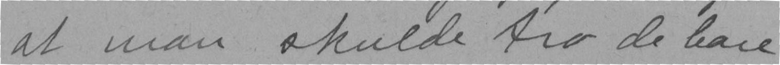at man skulde fro de bare
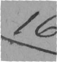16
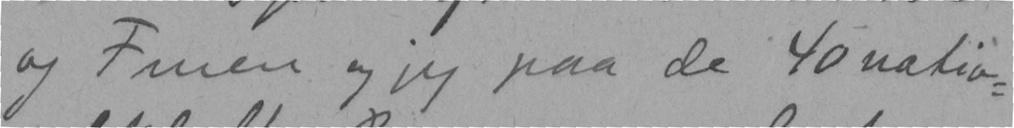og Fruen og jeg paa de 40 natio-
